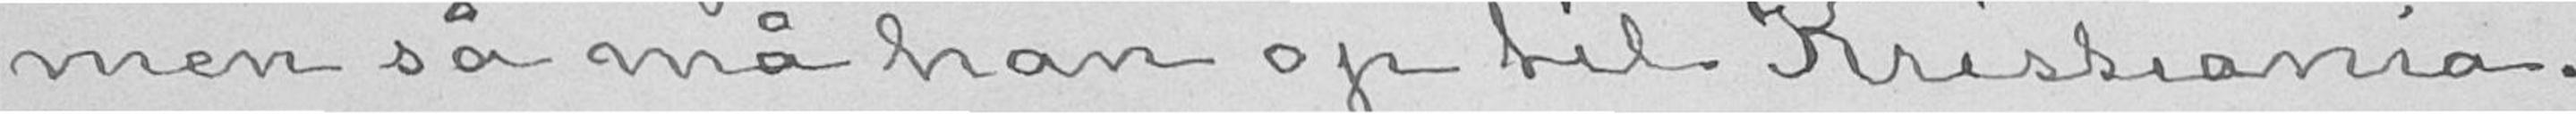men så må han op til Kristiania.
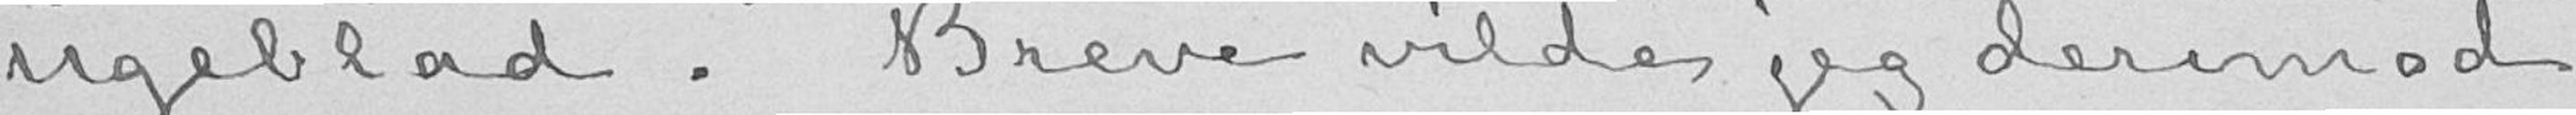ugeblad. Breve vilde jeg derimod
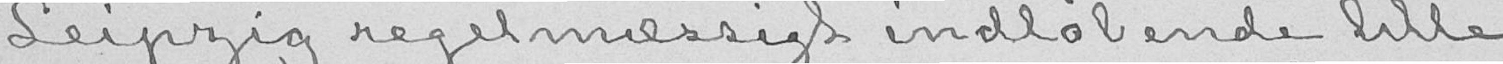Leipzig regelmæssigt indløbende lille
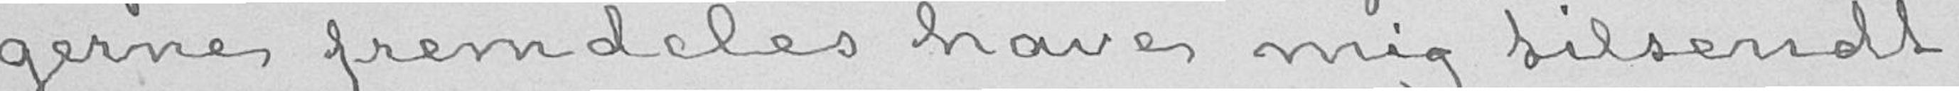gerne fremdeles have mig tilsendt,
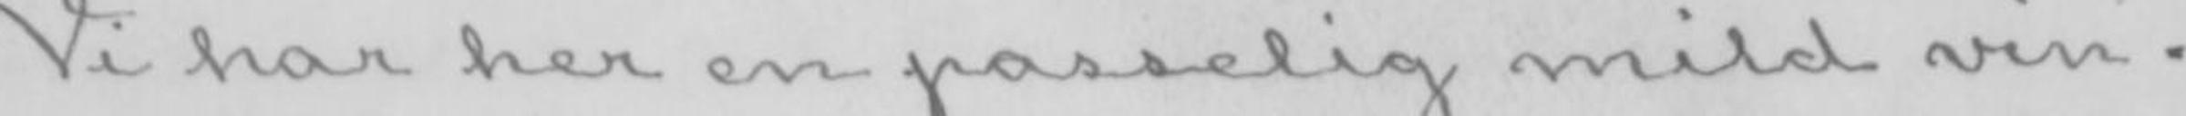Vi har her en passelig mild vin-
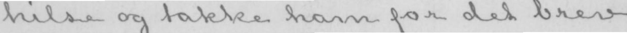hilse og takke ham for det brev,
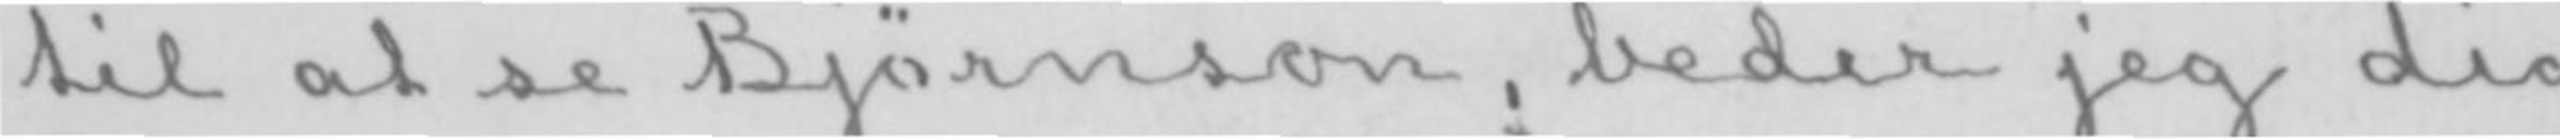til at se Bjørnson, beder jeg dig
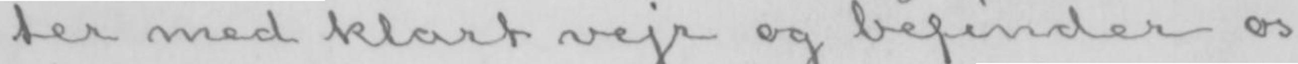ter med klart vejr og befinder os
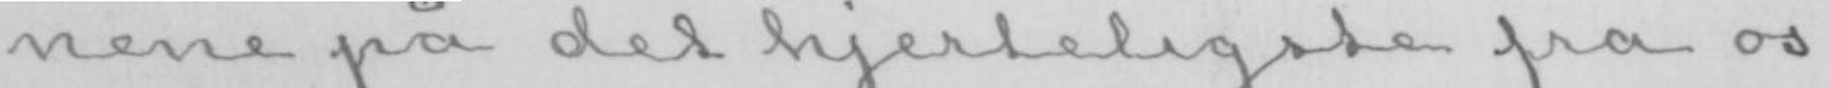nene på det hjerteligste fra os
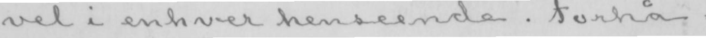vel i enhver henseende. Forhå-
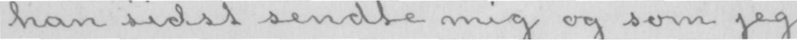han sidst sendte mig og som jeg
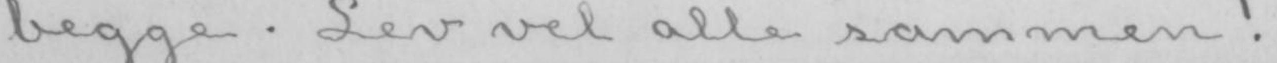begge. Lev vel alle sammen!
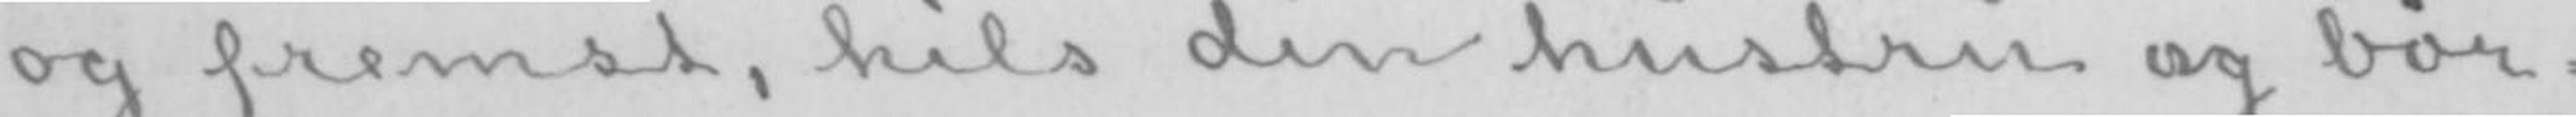og fremst, hils din hustru og bør-
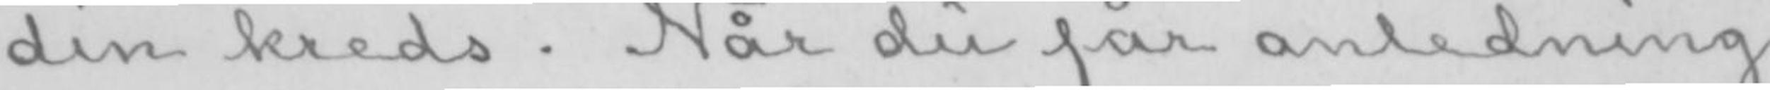din kreds. Når du får anledning
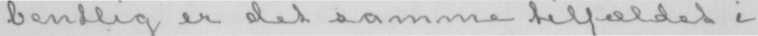bentlig er det samme tilfældet i
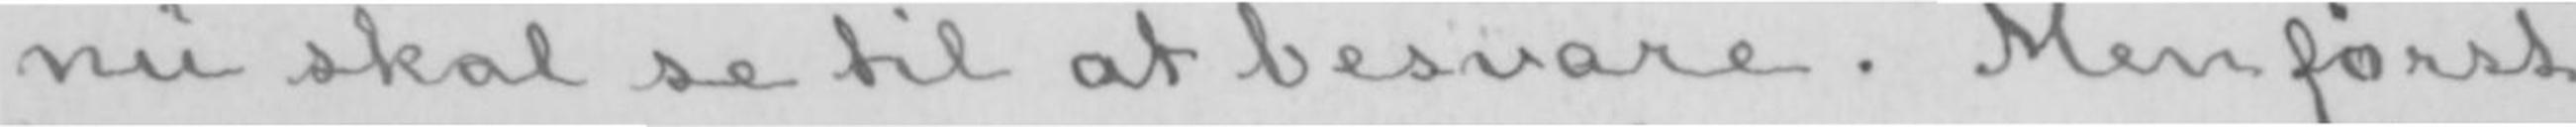nu skal se til at besvare. Men først
